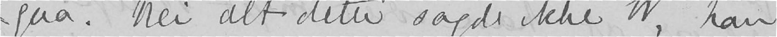gaa. Nei alt dette sagde ikke W, han
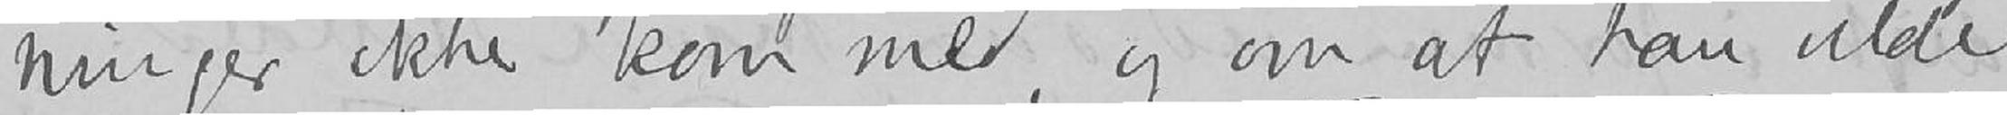ninger ikke kom med, og om at han vilde
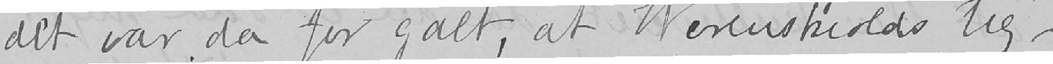det var da for galt, at Werenskiolds teg-
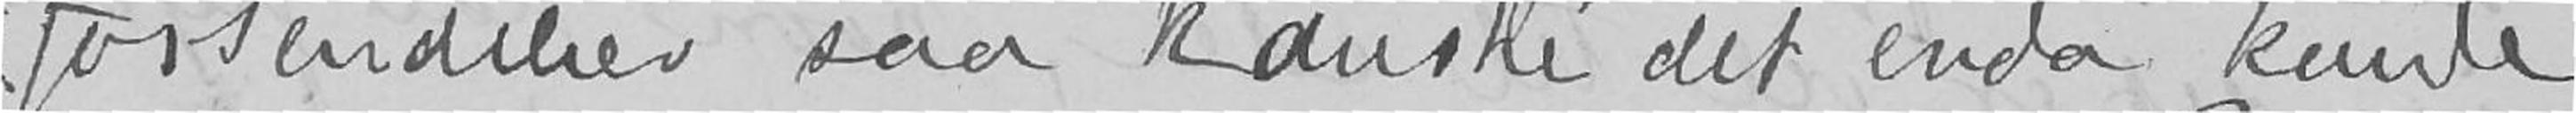forsendelser saa kanske det enda kunde
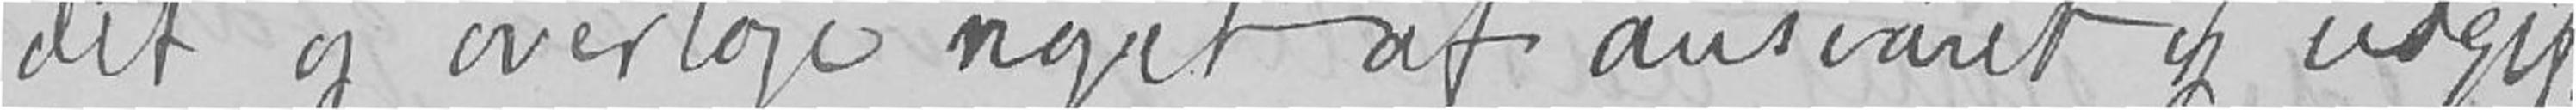det og overtage noget af ansvaret og udgif
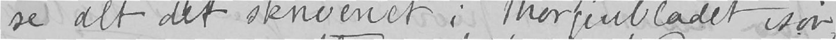se alt det skriveriet i Morgenbladet isøn-
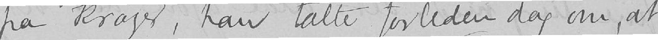fra Krøyer, han talte forleden dag om, at
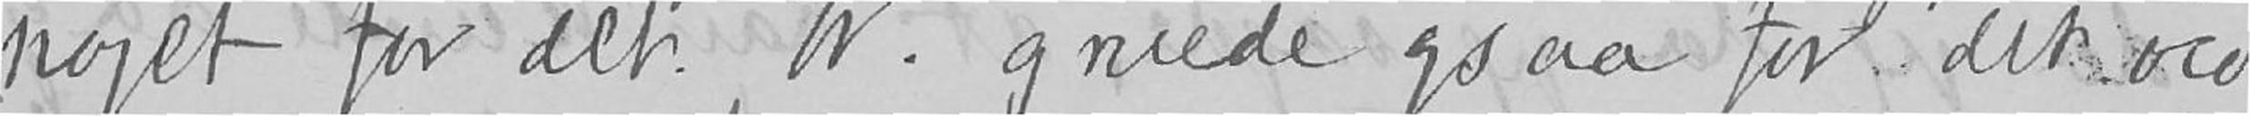noget for det. W. gruede ogsaa for det øco
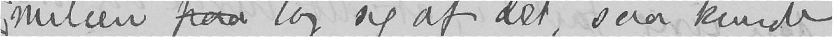mitéen paa tog sig af det, saa kunde
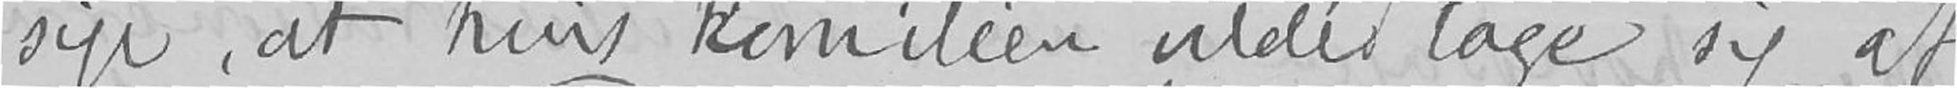sige, at hvis komitéen vilde tage sig af
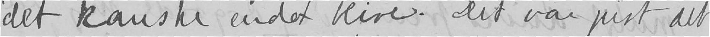det kanske enda blive. Det var just det
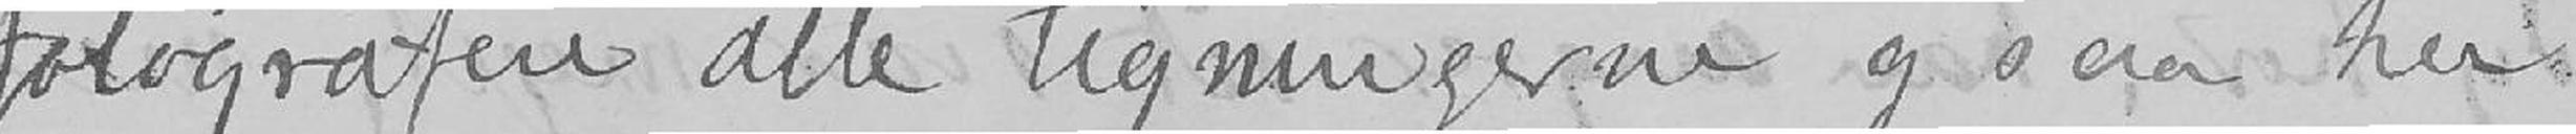fotografere alle tegningerne ogsaa her
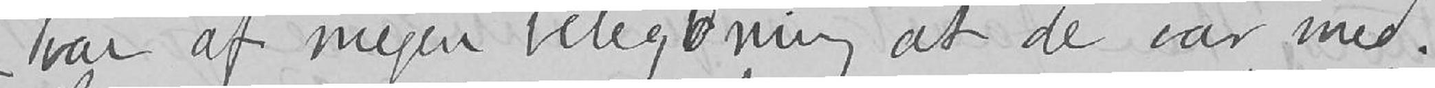var af megen betydning at de var med.
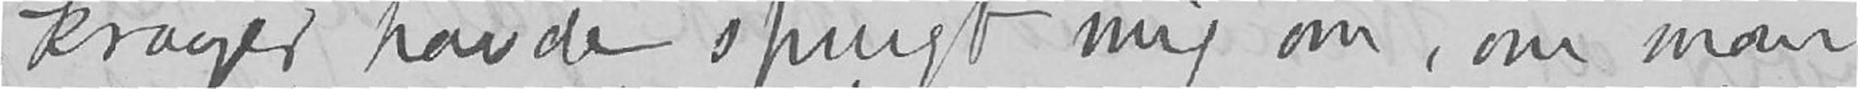Krøyer havde spurgt mig om, om man
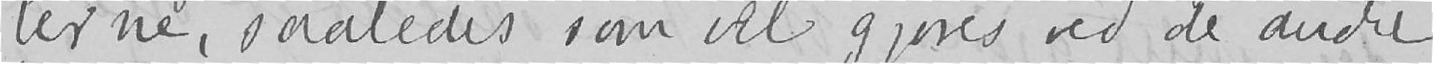terne, saaledes som vel gjøres ved de andre
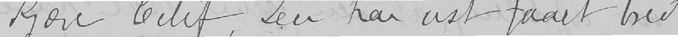Kjære Eilif, Du har vist faaet brev
